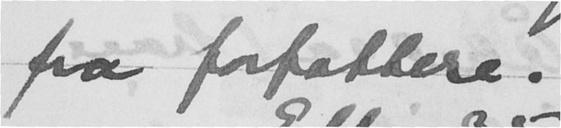fra forfattere.
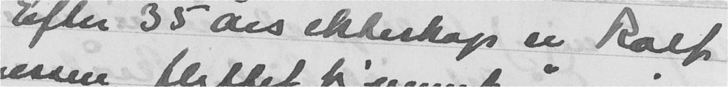Efter 35 års ekteskap er Rolf
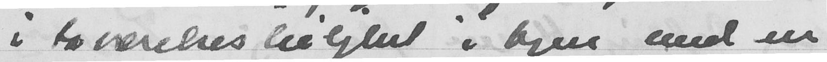i toværelses leilighet i byen med en
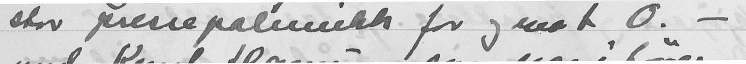stor pressepolemikk for og mot O. -
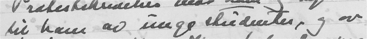til ham av unge studenter, og av
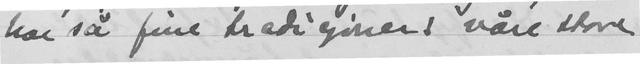har så fine tradisjoner! våre store
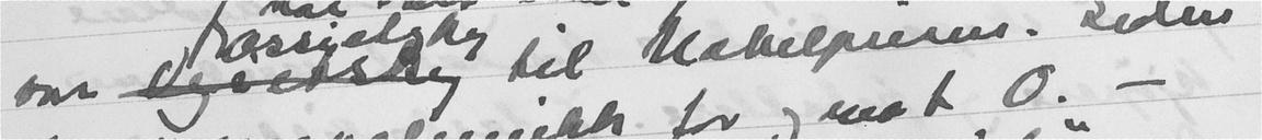om  Ossietzky til Nobelprisen. Siden
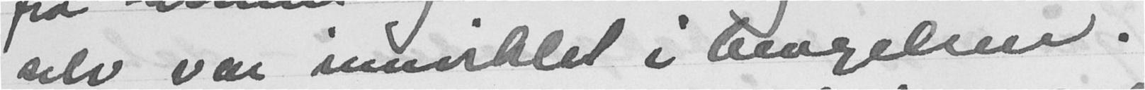selv var innviklet i bevegelsen.
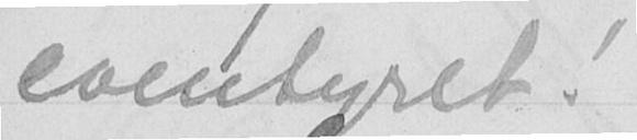eventyret!
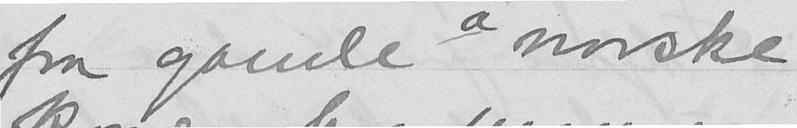fra gamle norske
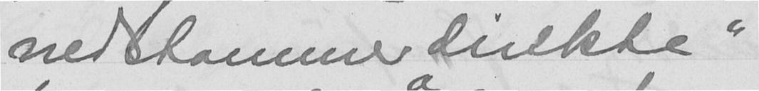nedstammer "direkte"
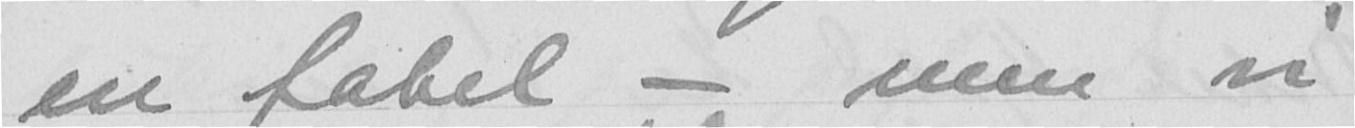en fabel - men vi
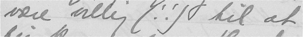være villig (!!) til at
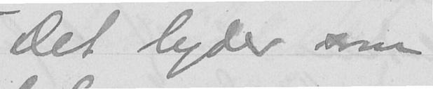Det lyder som
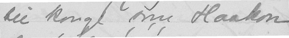bli konge som Haakon
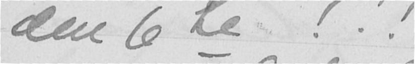den 6te!!!
